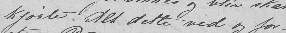kjørte. Alt dette ved og for-
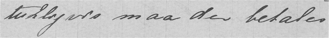turligvis maa der betales
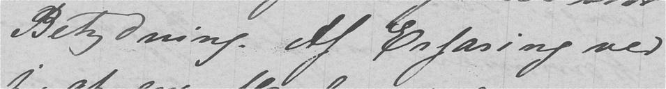Betydning. Af Erfaring ved
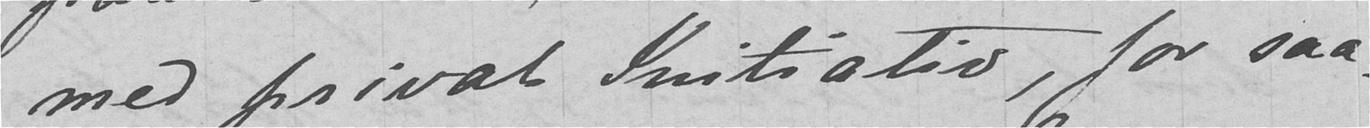med privat Initiativ, for saa
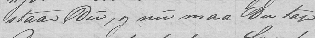staar Du, og nu maa Du tage
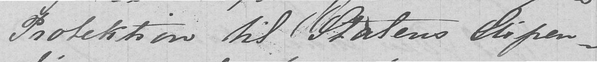Protektion til Statens Stipen-
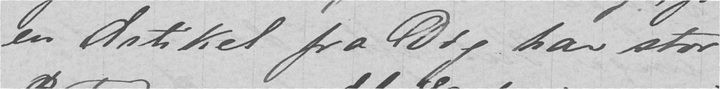en Artikel fra Dig har stor
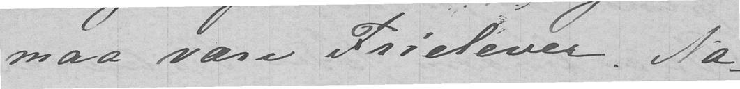maa være Frielever. Na-
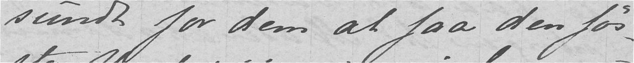sundt for dem at faa den før-
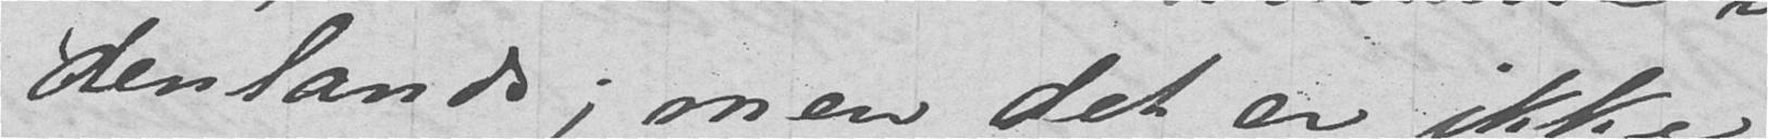denlande; men det er ikke
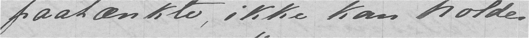paatænkte, ikke kan holdes
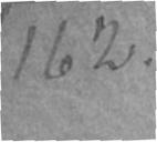162.
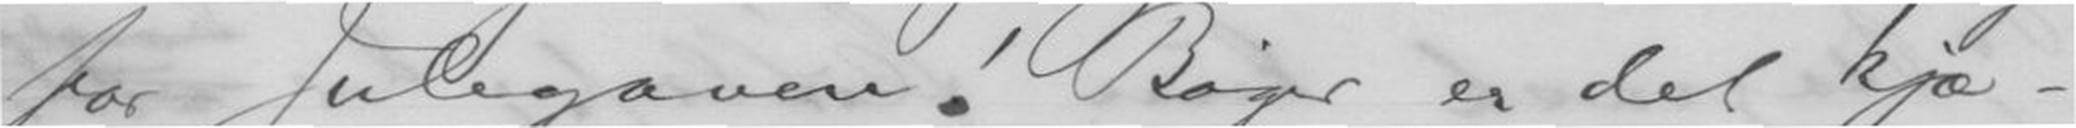for Julegaven! Bøger er det kjæ-
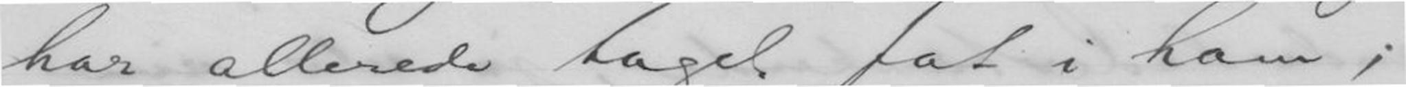har allerede taget fat i ham;
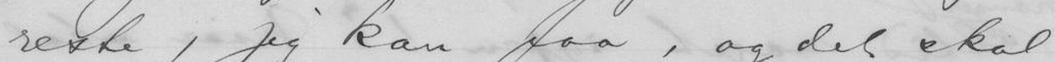reste, jeg kan faa, og det skal
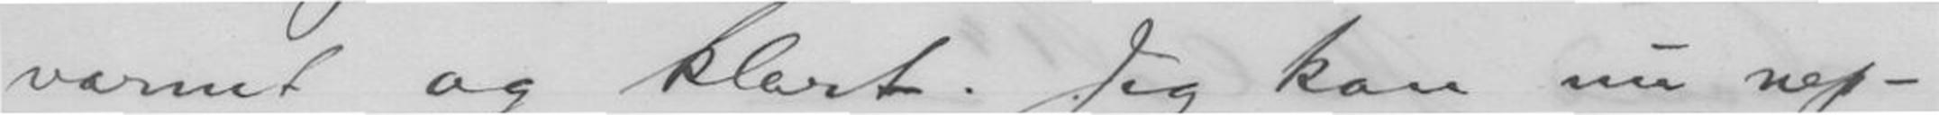varmt og klart. Jeg kan nu nep-
